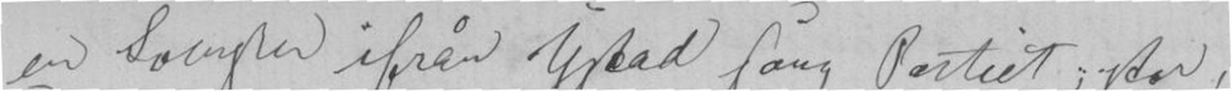en Svensker ifrån Ystad sang Partiet; stor,
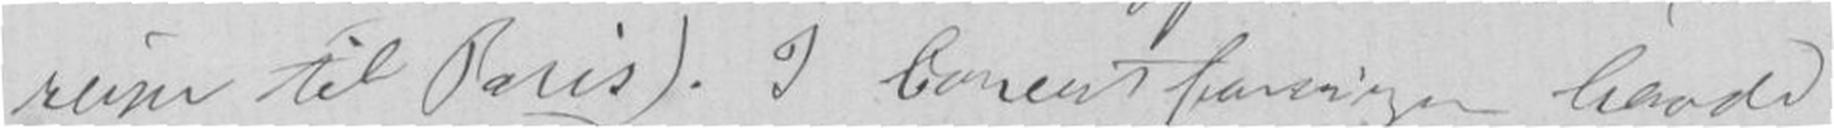reiser til Paris). I Concertforeningen havde
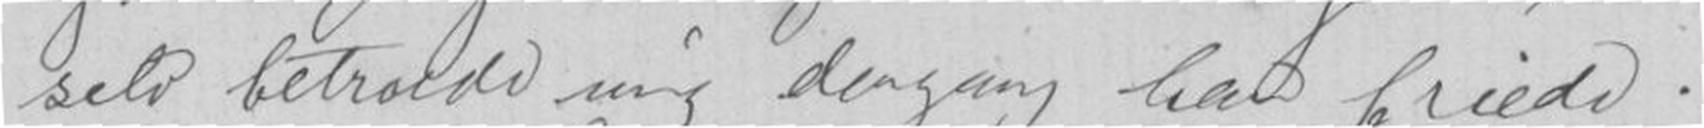selv betroede mig dengang han friede.
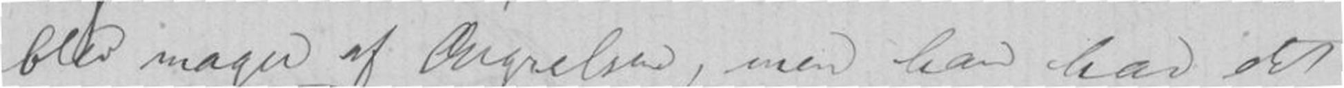blev mager af Ærgrelse, men han har det
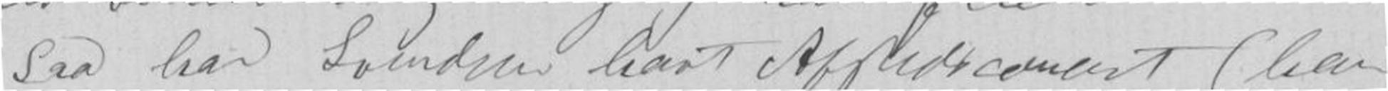Saa har Svendsen havt Afskedsconcert (han
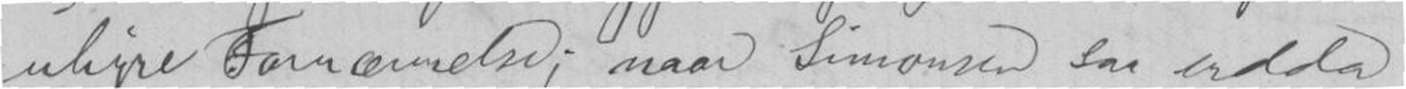uhyre Fornærmelse; naar Simonsen saa endda
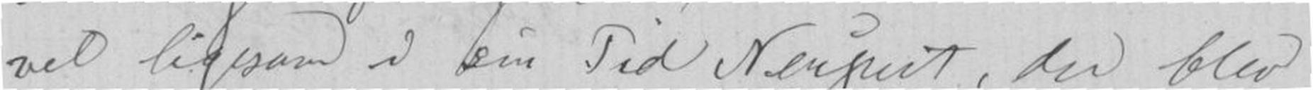vel ligesom i sin Tid, Neupert, der blev
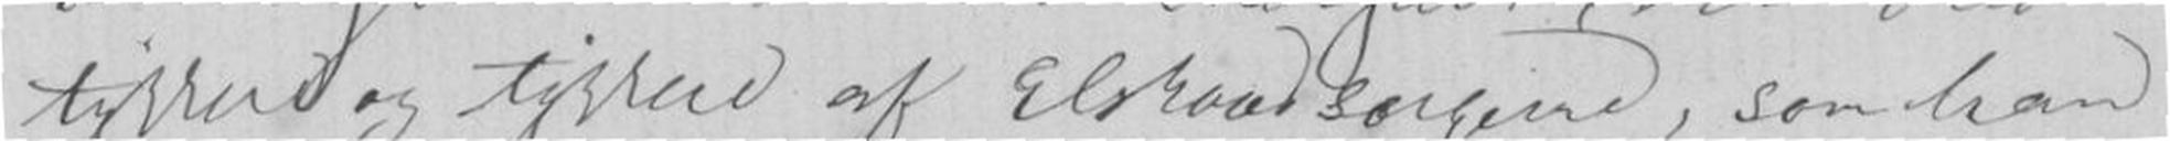tykkere og tykkere af Elskovssorgerne, som han
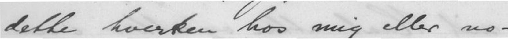dette hverken hos mig eller no-
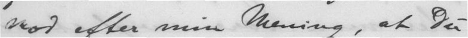mod efter min Mening, at Du
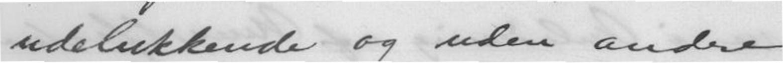udelukkende og uden andre
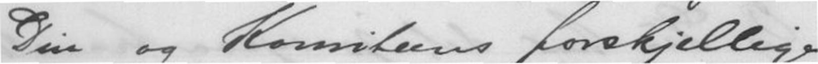Din og Komiteens forskjellige
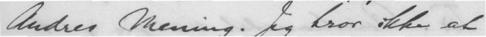Andres Mening. Jeg tror ikke at
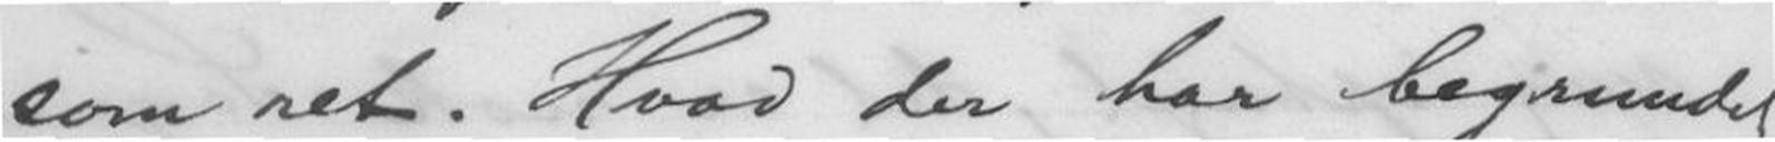som ret. Hvad der har begrundet
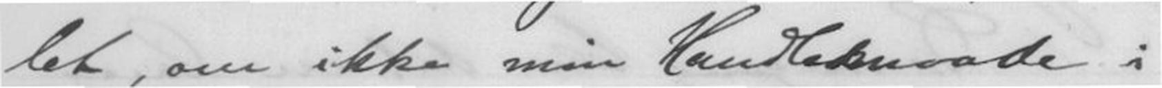let, om ikke min Handlemaade i
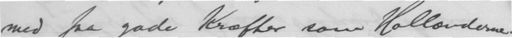med saa gode Kræfter som Hollænderne.
